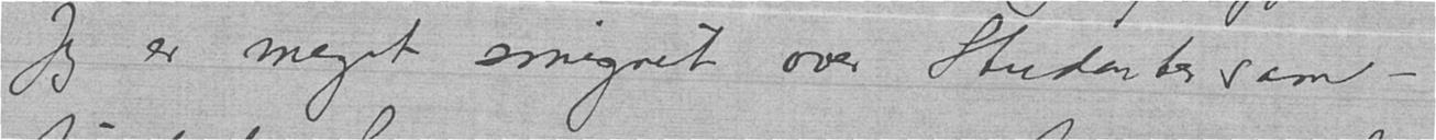Jeg er meget smigret over Studentersam-
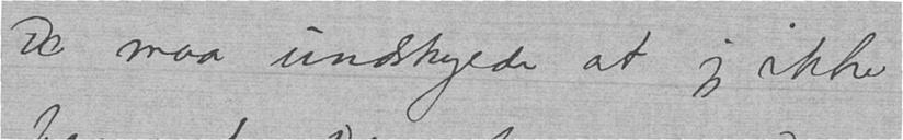De maa undskylde at jeg ikke
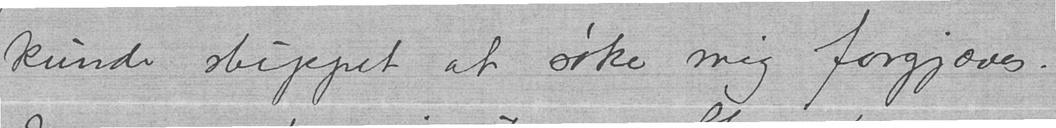kunde sluppet at søke mig forgjæves.
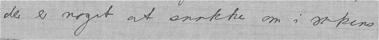der er noget at snakke om i sakens
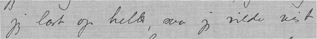jeg læst op heller, saa jeg vilde vist
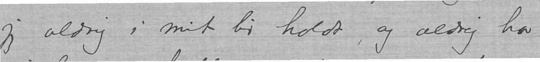jeg aldrig i mit liv holdt, og aldrig har
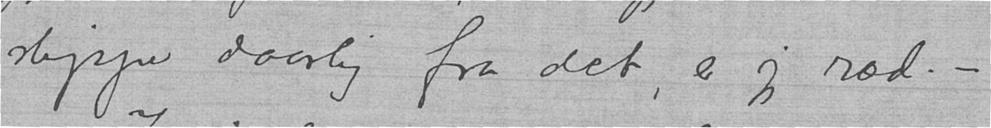slippe daarlig fra det er jeg ræd. -
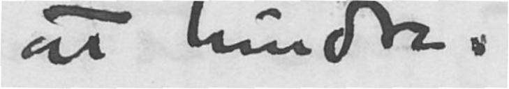at hindre.
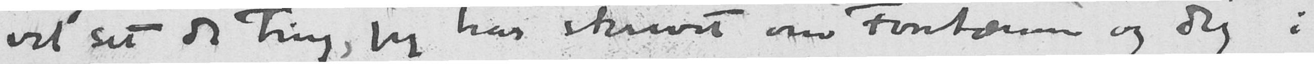vel set de ting jeg har skrevet om "Fontænen" og dig i
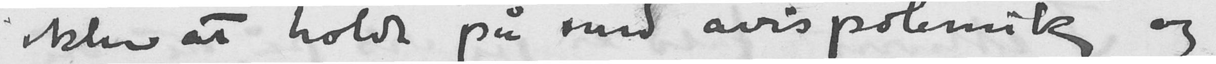ikke at holde på med avispolemik og
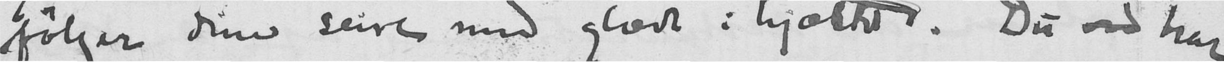følger dine seire med glæde i hjærtet. Du ved har
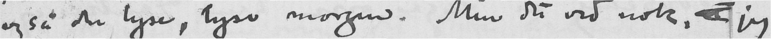også den lyse, lyse morgen. Men du ved nok, at jeg
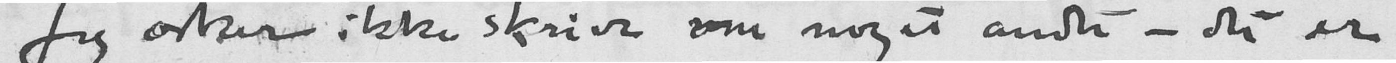Jeg orker ikke skrive om noget andet - det er
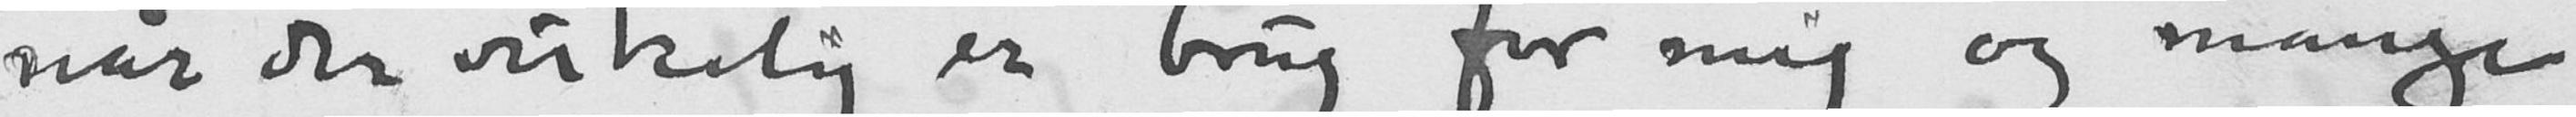når der virkelig er brug for mig og mange
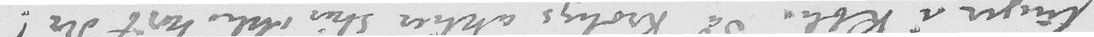linger i Kbh. Så Krohgs aktier står ikke høit der!
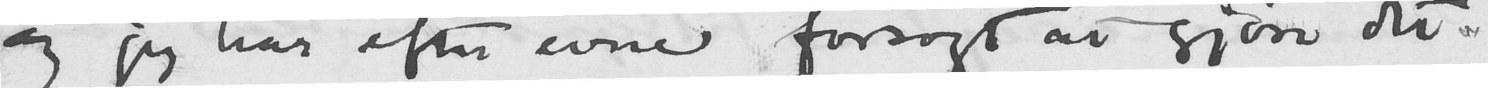og jeg har efter evne forsøgt at gjøre det.
